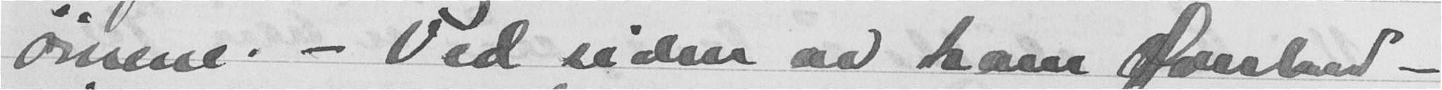øinene. - Ved siden av ham Øverland -
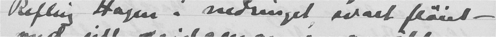Refling Hagen i nedringet, svart fløist -
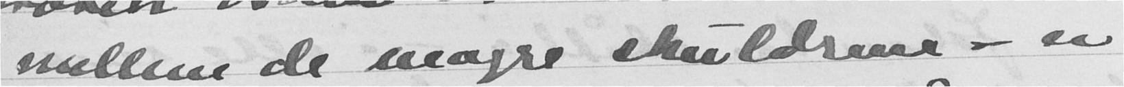mellom de magre skuldrene - er
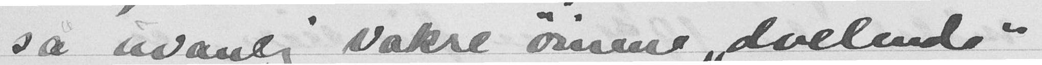så uvanlig vokse øinene, "dvelende"
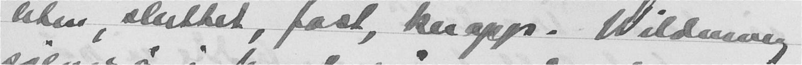liten, sluttet, fast, knapp. Wildennig
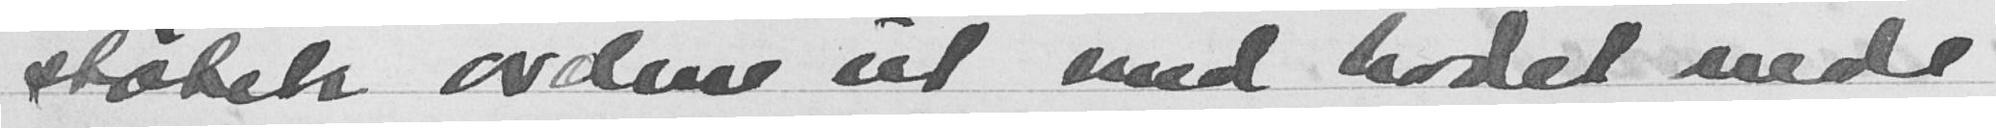støtet av dem ut med hodet nede
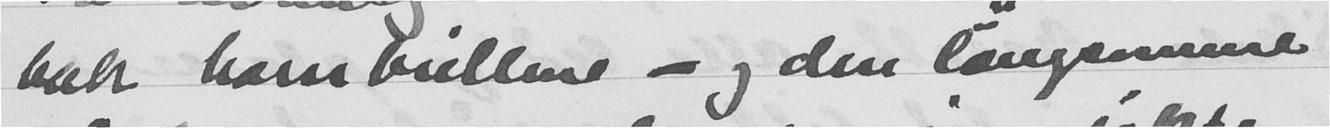bak hornbrillene - og den langsomme
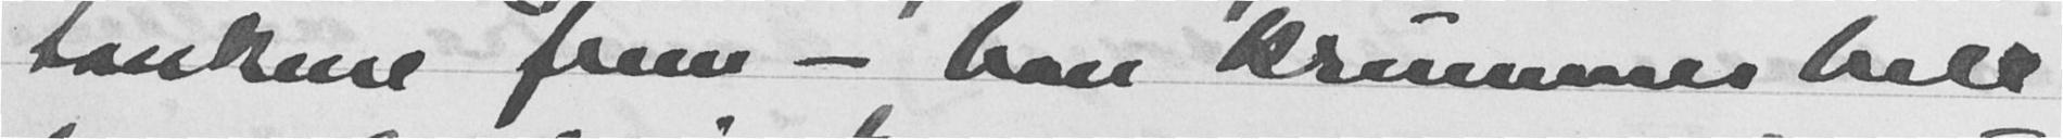tankene frem - han krummer helt
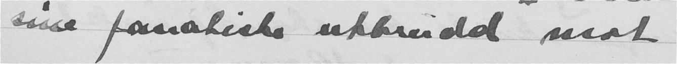sine fantastiske utbrudd mot
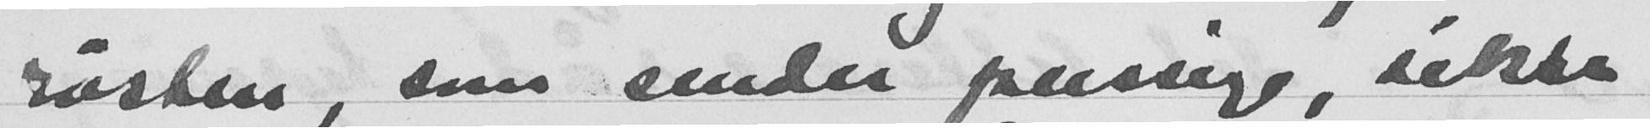røsten, som sender pussige, søkte
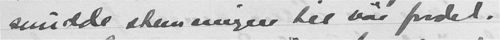snudde stemningen til vår fordel.
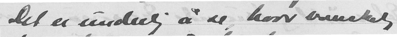Det er underlig å se, hvor vanskelig
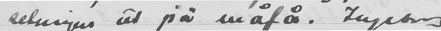setninger ut på måfå. Ingeborg
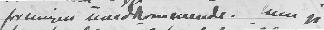foreningen uvedkommende, men jeg
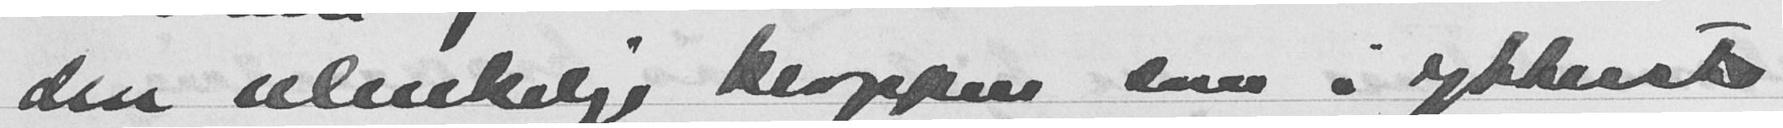den utenkelige kroppen, som i ytterste
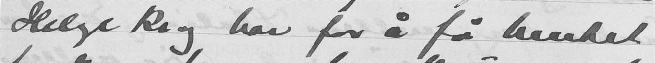Helge Krog har for å få hentet
